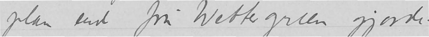plan end fru Wettegreen gjorde.
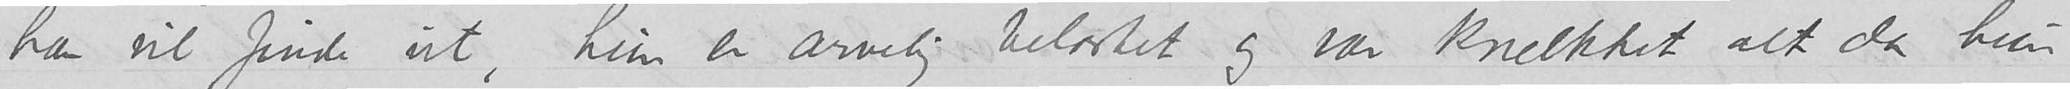han vil finde ut, hun er arvelig belastet og var knelkket alt da hun
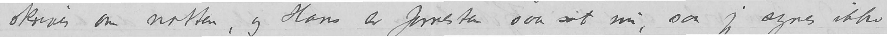skrives om natten , og Hans er forresten saa søt nu, saa jeg syns ikke
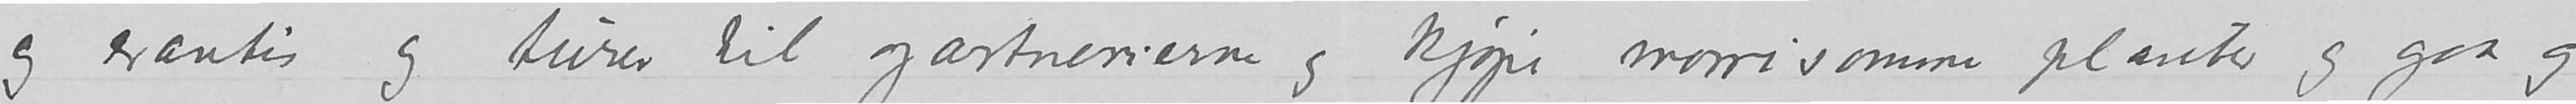og erantis og turer til gartnerierne og kjøpe morrisomme planter og gaa
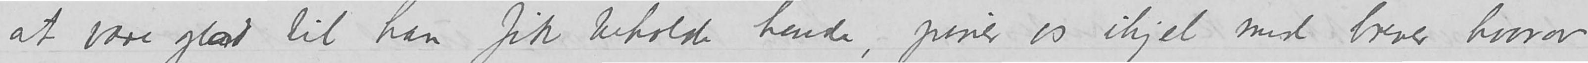at være glad til han fik beholde hende, piner os i hjel med brever hvorav
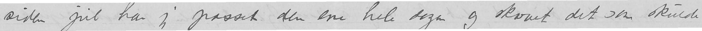siden jul har jeg passet den ene hele dagen og skrevet det som skulde
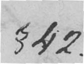§ 42.
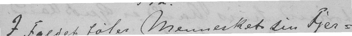I Faldet føler Mennesket sin Fjer-
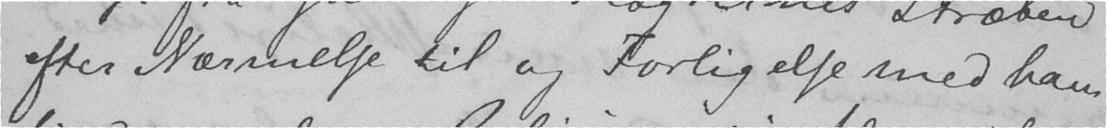efter Nærmelse til og Forligelse med ham
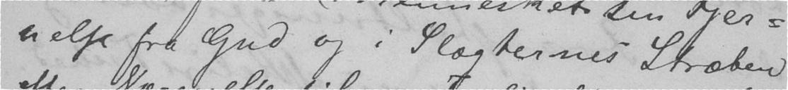nelse fra Gud og i Slægternes Stræben
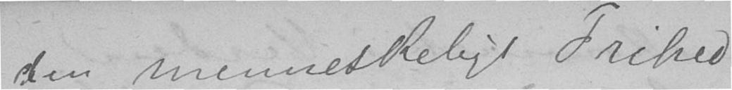den menneskelige Frihed
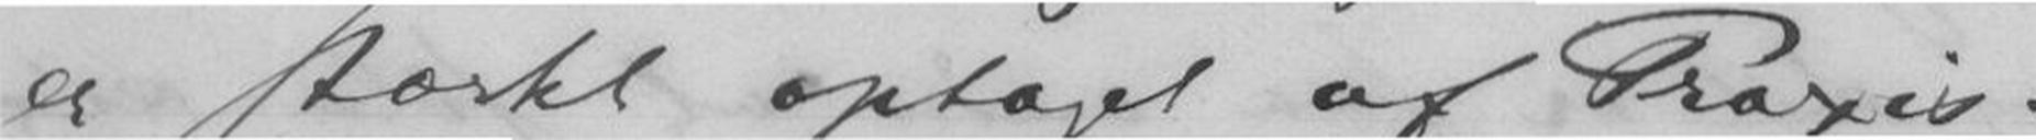er stærkt optaget af Praxis.
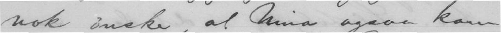nok ønske, at Nina ogsaa kom
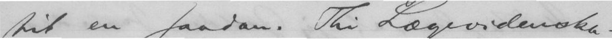til en saadan. Thi Lægevidenska-
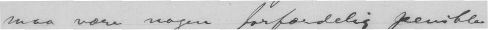maa være nogen forfærdelig penible
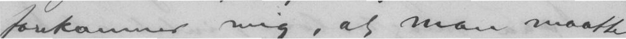forekommer mig, at man maatte
